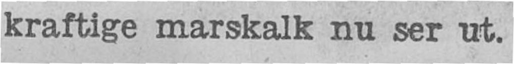kraftige marskalk nu ser ut.
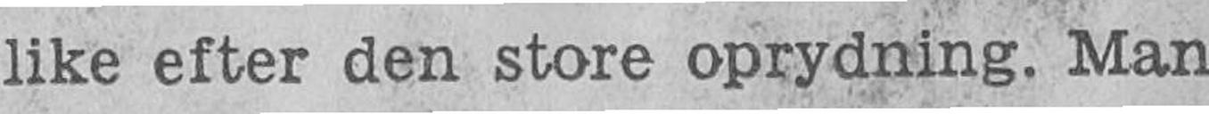like efter den store oprydning. Man
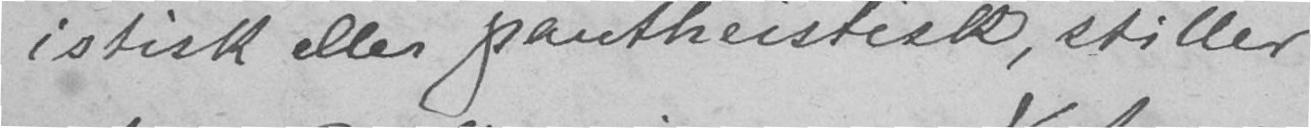istisk eller pantheistisk, stiller
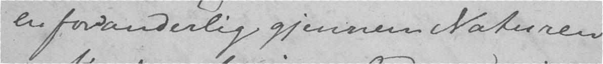en foranderlig gjennem Naturen
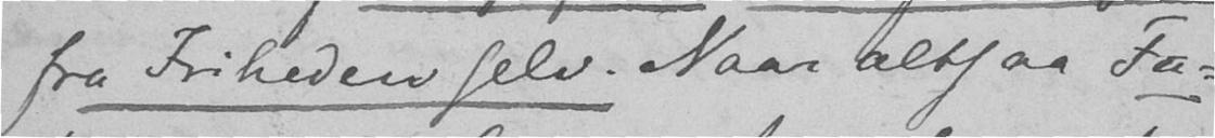fra Friheden selv. Naar altsaa Fa-
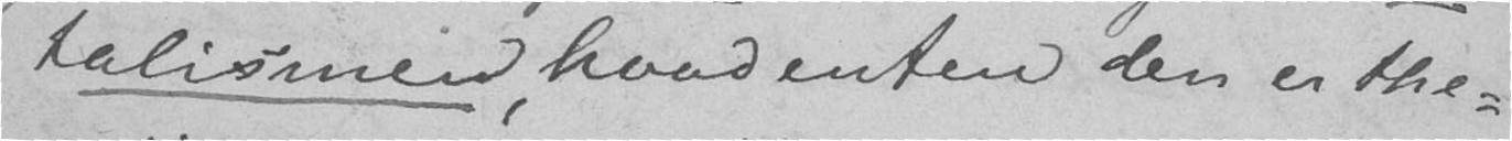talismen, hvad enten den er the-
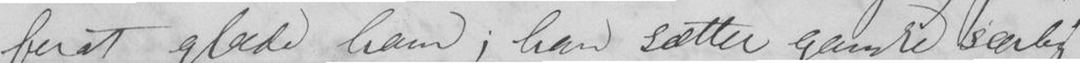forat glæde ham; han sætter ganske særlig
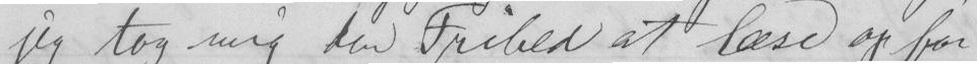jeg tog mig den Frihed at læse op for
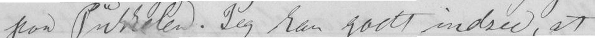paa Pukkelen. Jeg kan godt indsee, at
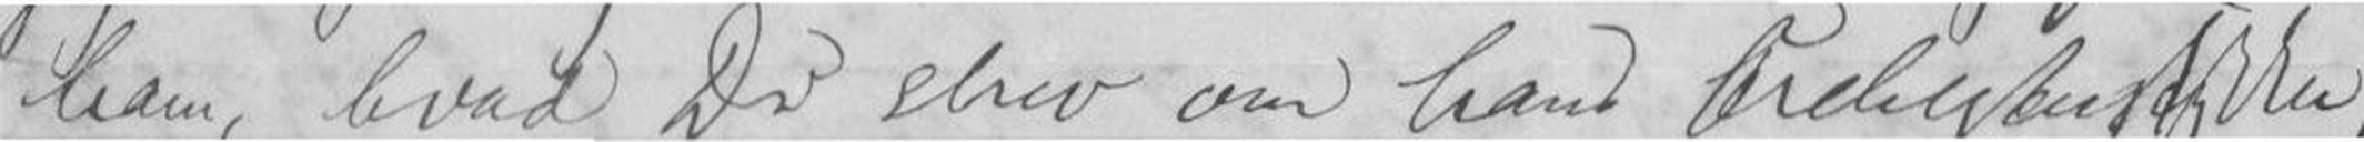ham, hvad Du skrev om hans Orchesterstykke,
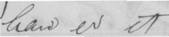han er et
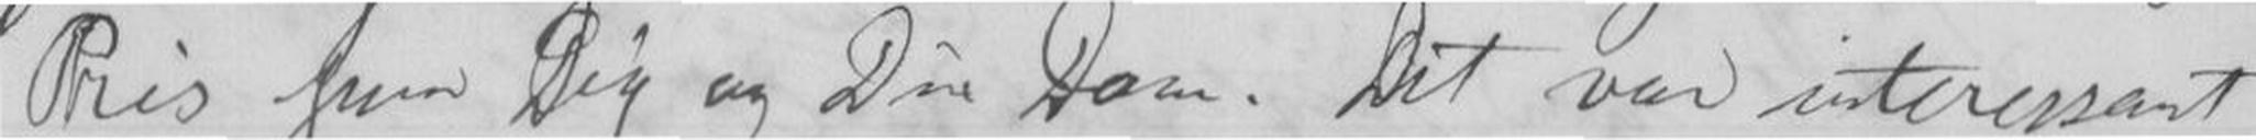Pris paa Dig og Din Dom. Det var interessant
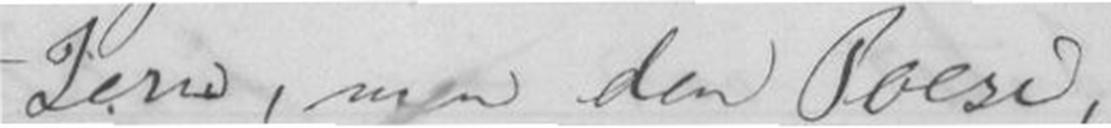Jern, men den Poesi,
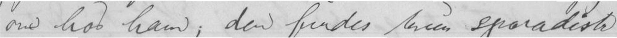om hos ham; der findes kun sporadisk
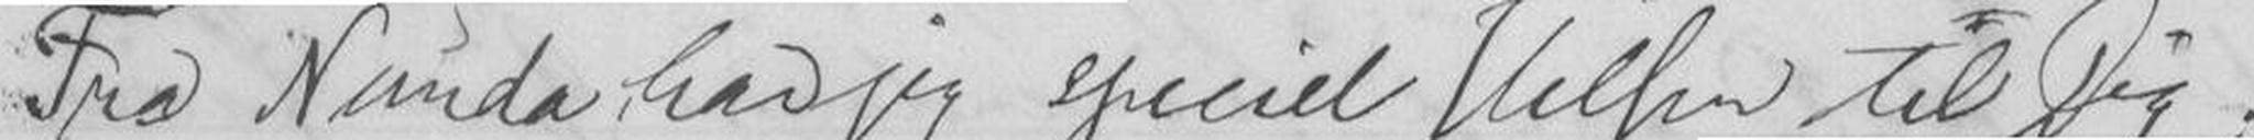Fra Neruda, har jeg speciel Hilsen til Dig;
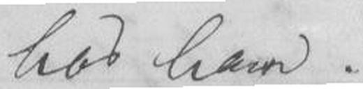hos ham.
